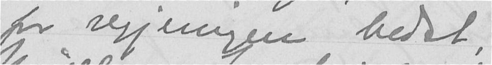for regjeringen bedst,
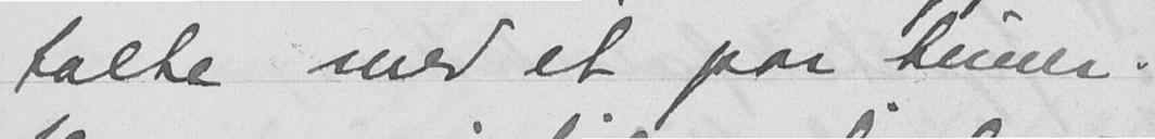talte med et par timer.
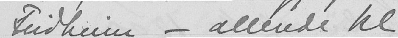Fridheim - allerede kl
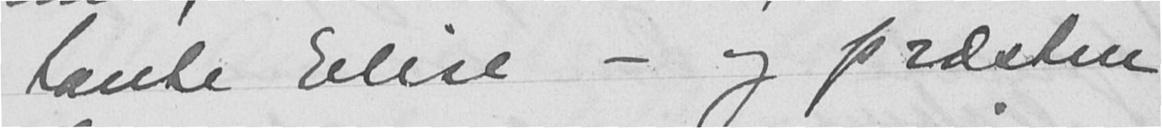tante Elise - og præsten
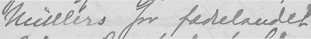Müllers for fædrelandet
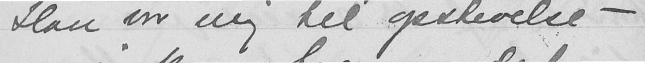Han var mig til opstivelse -
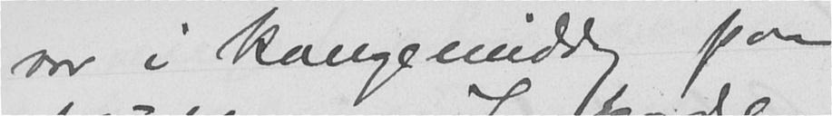var i kongemiddag paa
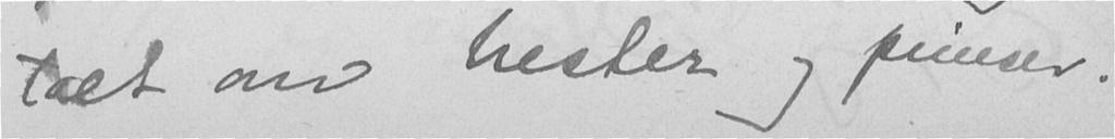talt om hester og prinser.
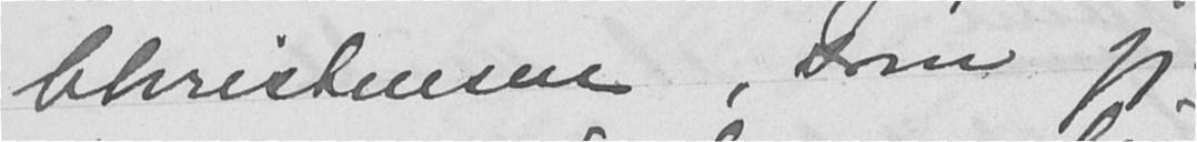Christensen, som jeg
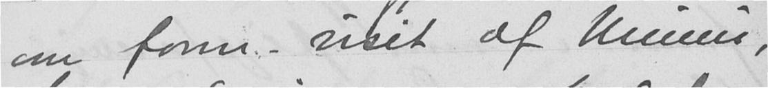om form. visit af Minni,
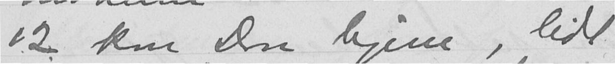12 kom Don hjem, lidt
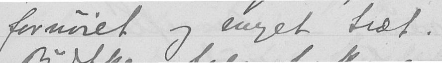fornøiet og meget træt.
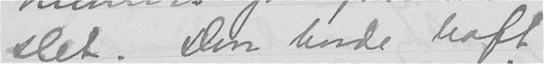slet. Don havde haft
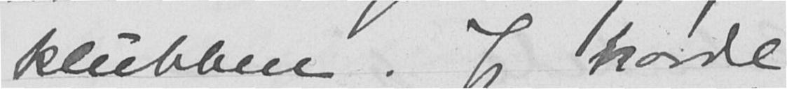klubben. Jeg havde
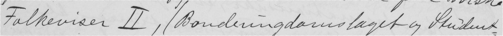Folkeviser 2, (Bondeungdomslaget og Student-
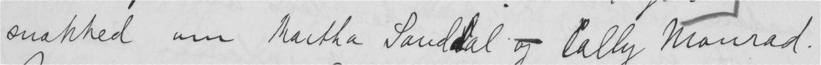snakked om Martha Sandal og Cally Monrad.
